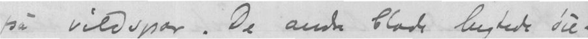på vildspor. De andre blade lugtede øie-
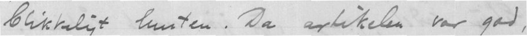blikkeligt lunten. Da artikelen var god,
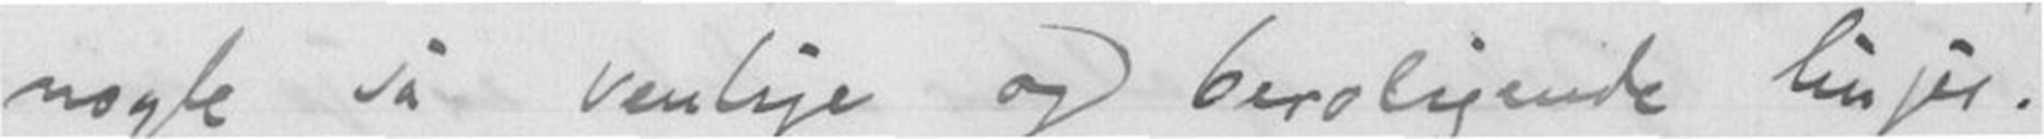nogle så venlige og beroligende linjer.
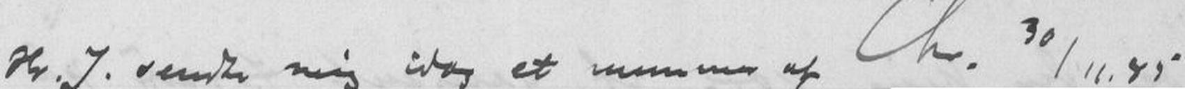Hr. I. sendte mig idag et nummer af Chr. 30/11.85
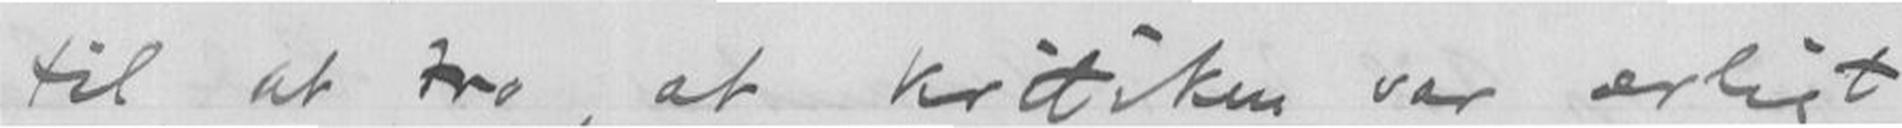til at tro, at kritiken var ærligt
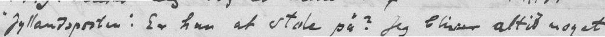"Jyllandsposten". Er han at stole på? Jeg bliver altid noget
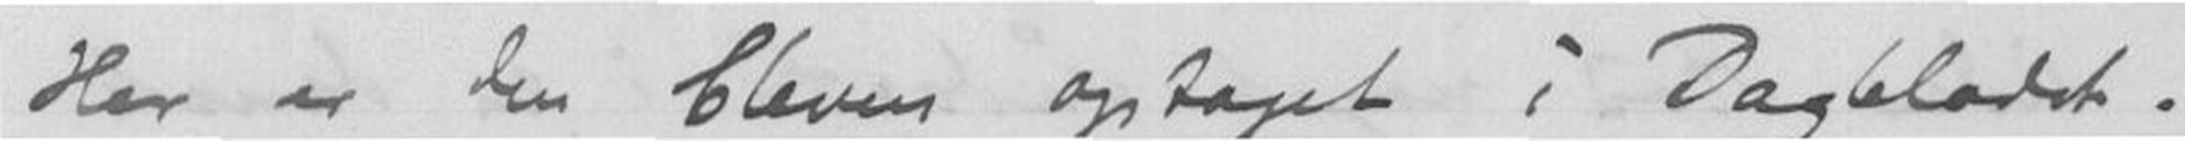Her er den bleven optaget i Dagbladet.
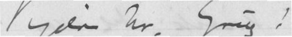Kjære hr. Grieg!
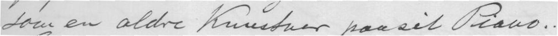som en ældre Kunstner paa sit Piano.
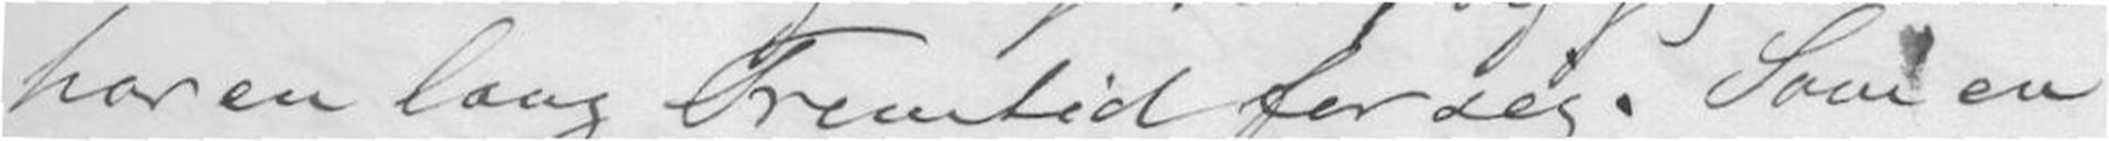har en lang Fremtid for sig. Som en
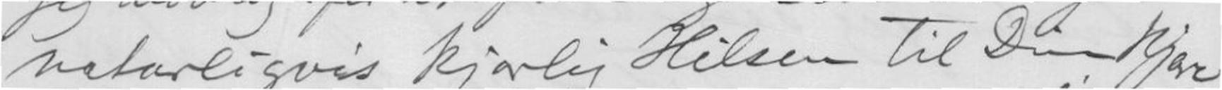naturligvis kjærlig Hilsen til Din kjære
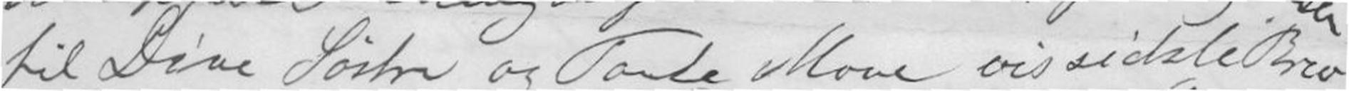til Dine Søstre og Tante Mone vis sidste Brev
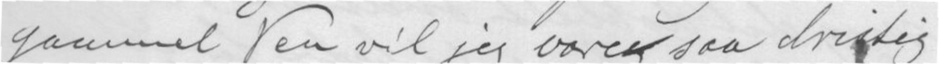gammel Ven vil jeg være saa dristig
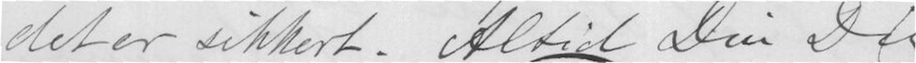det er sikkert. Altid Din Dig
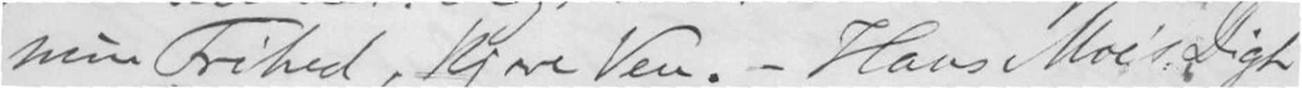min Frihed, Kjære Ven. - Hans Moe's Digt
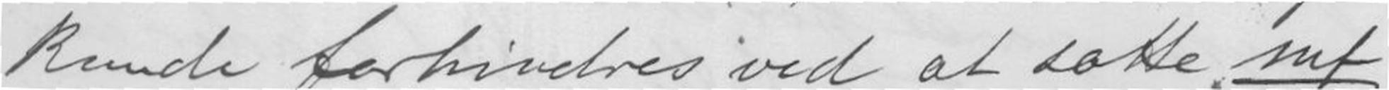kunde forhindres ved at sætte mf
